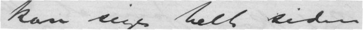jeg kan sige helt siden
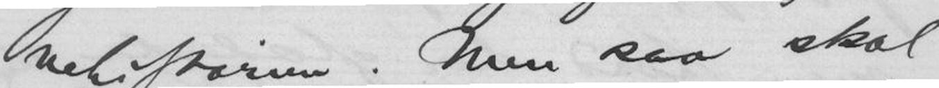vehistorien. Men saa skal
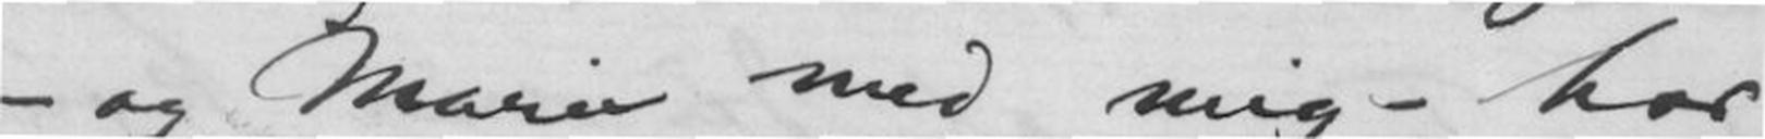- og Marie med mig - har
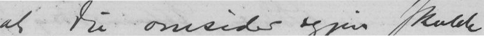at du omsider igjen skulde
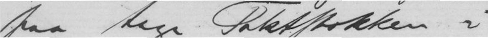faa tage Taktstokken i
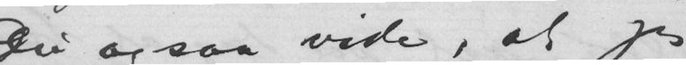Du ogsaa vide, at jeg
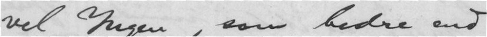vel Ingen, som bedre end
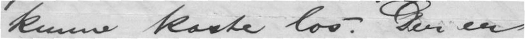kunne kaste los. Der er
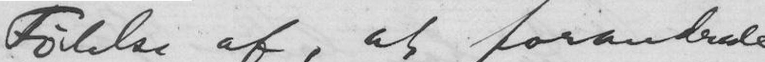Følelse af, at forandrede
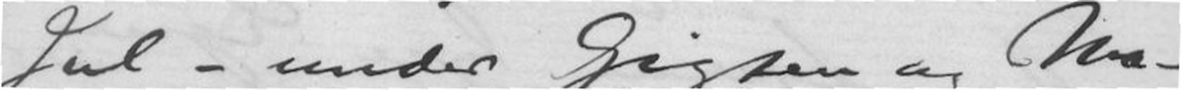Jul - under Gigten og Ma-
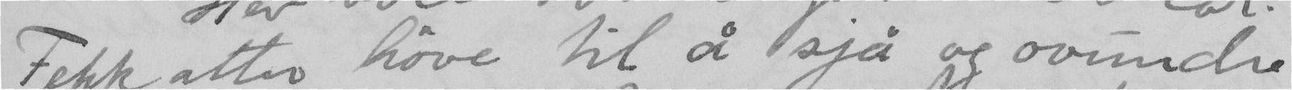Fekk atter höve til å sjå og ovundre
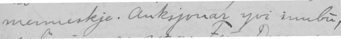menneskje. Auksjonar yvi innbu,
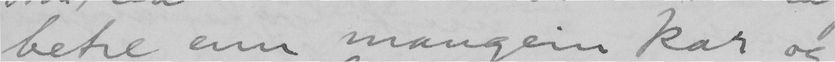betre enn mang ein kar og
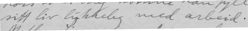sitt liv lukkelig med arbeid.
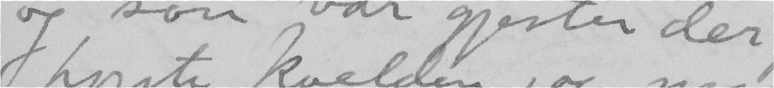og son var gjester der.
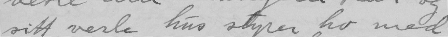sitt vesle hus styrer ho med
